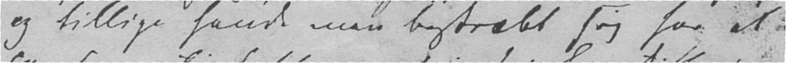og tillige havde man bestræbt sig for at
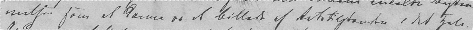melser som at danne os et Billede af Retstilstanden i det hele.
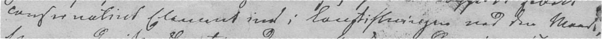conservativt Element ind i Lovstiftningen ved den Maade,
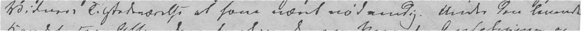Vidners Tilstedeværelse at have været nødvendig. Under den levende
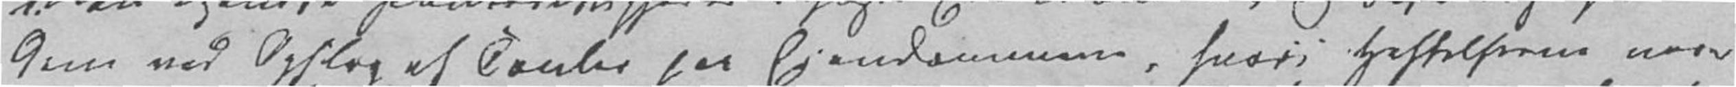dem ved Opslag af Tavler paa Eiendommene, hvori Heftelserne vare
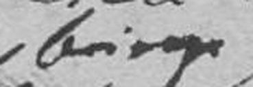bringe
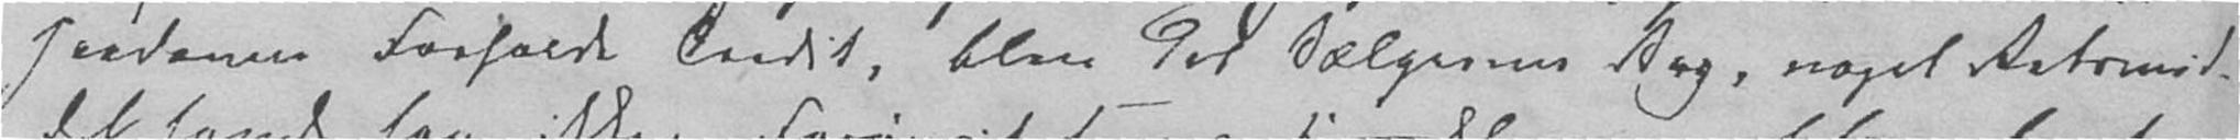saadanne Forholde Credit, blev det Sælgerens Sag, noget Retsmid-
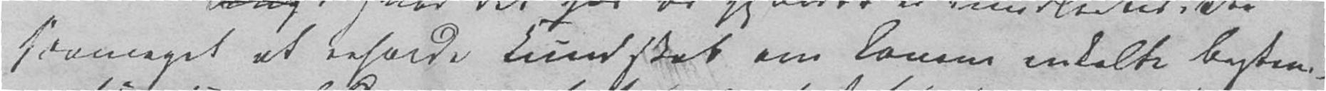saameget at erholde Kundskab om Lovens enkelte Bestem-
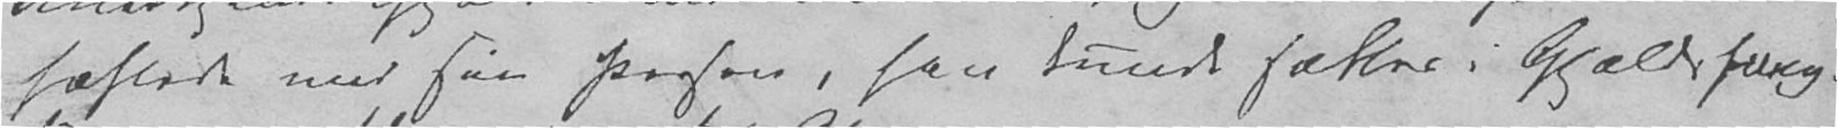hæftede med sin Person, han kunde sættes i Gjældsfeng-
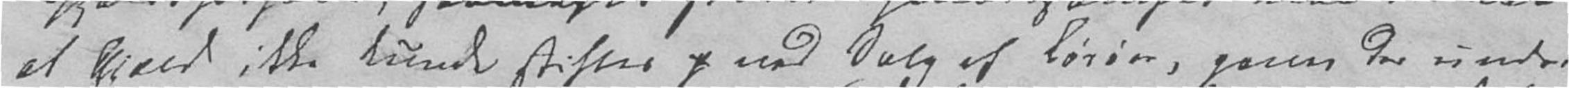at Gjeld ikke kunde stiftes ved Salg af Løsøre, gaves der under
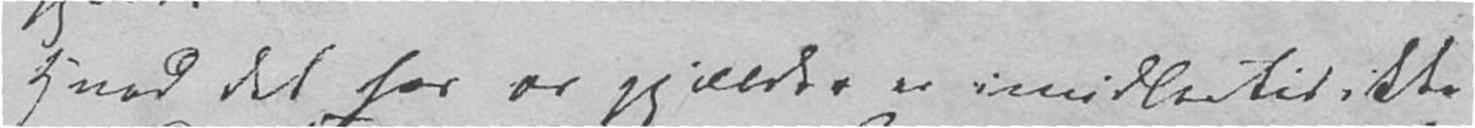Hvad det for os gjælder er imidlertid ikke
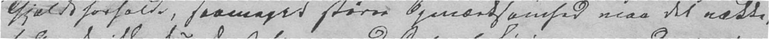Gjeldsforholde, saameget større Opmærksomhed maa det vække,
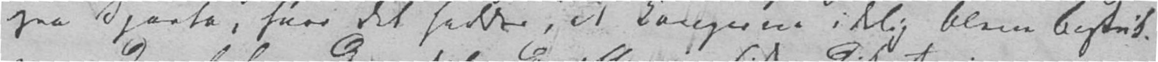fra Sparta, hvor det hedder, at Kongene idelig bleve bestuk-
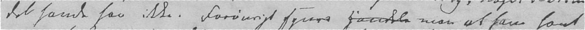del havde han ikke. Forøvrigt synes man at have havt
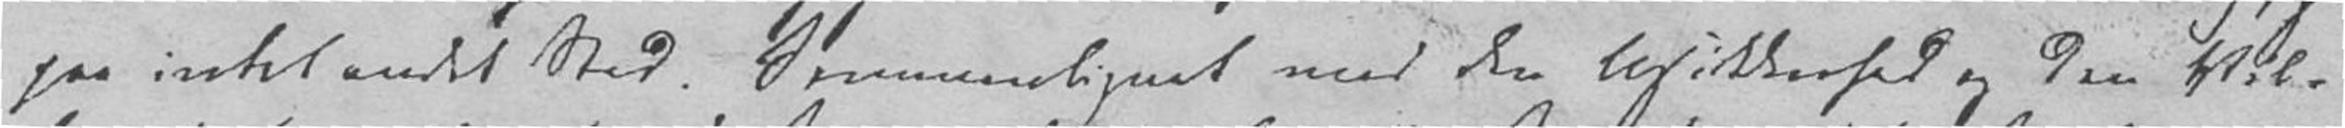paa intet andet Sted. Sammenlignet med den Usikkerhed og den Vil-
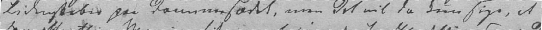Lidenskaber paa Dommersædet, men det vil da kun sige, at
